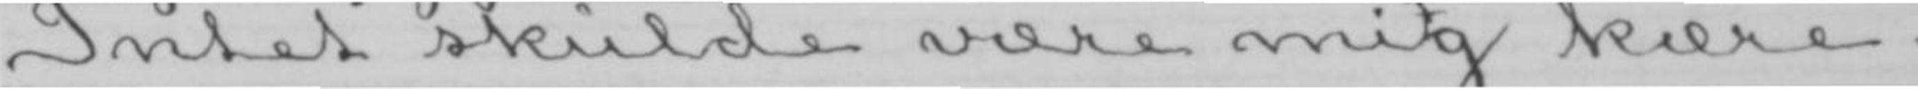Intet skulde være mig kære-
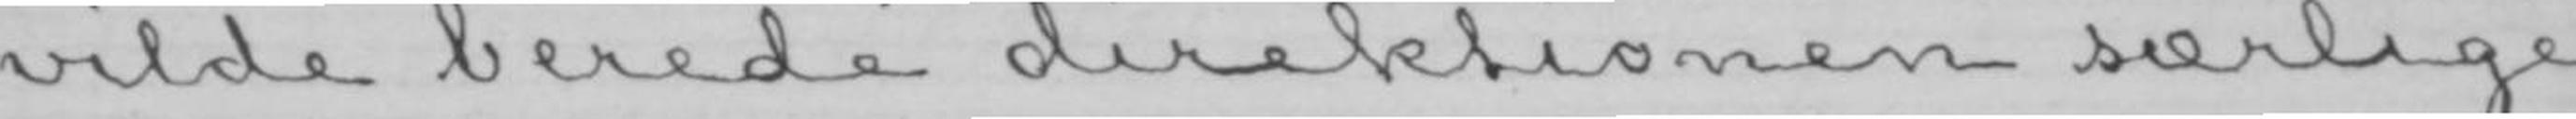vilde berede direktionen særlige
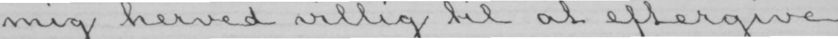mig herved villig til at eftergive
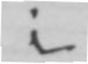i
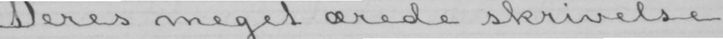Deres meget ærede skrivelse
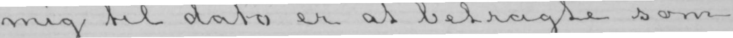mig til dato er at betragte som
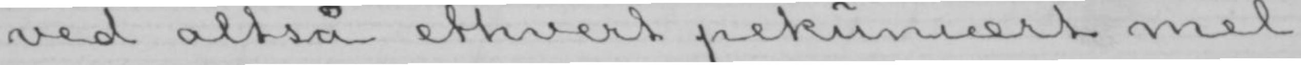ved altså ethvert pekuniært mel-
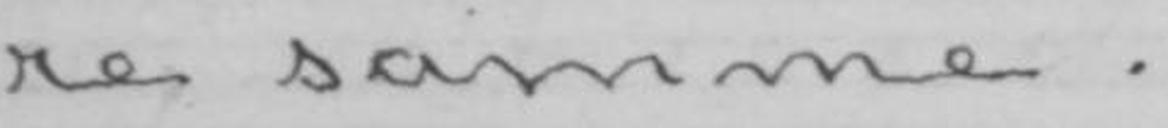re samme.
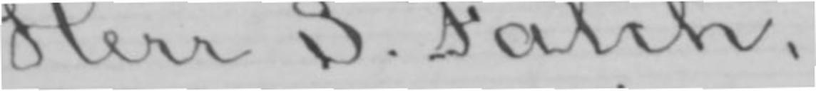Herr S. Falch,
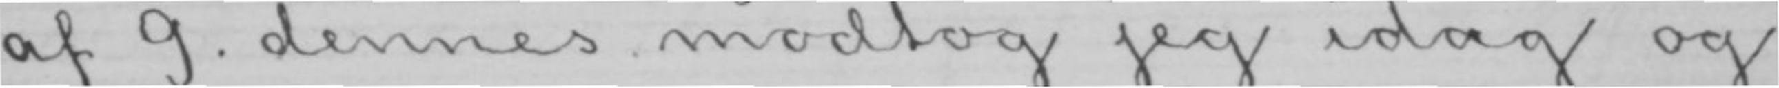af 9. dennes modtog jeg idag og
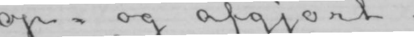op- og afgjort.
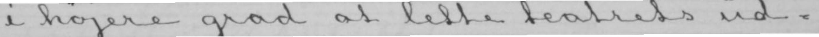i højere grad at lette teatrets ud-
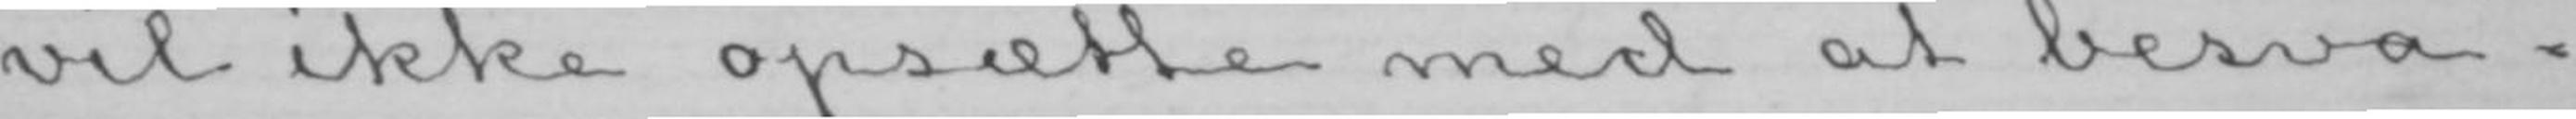vil ikke opsætte med at besva-
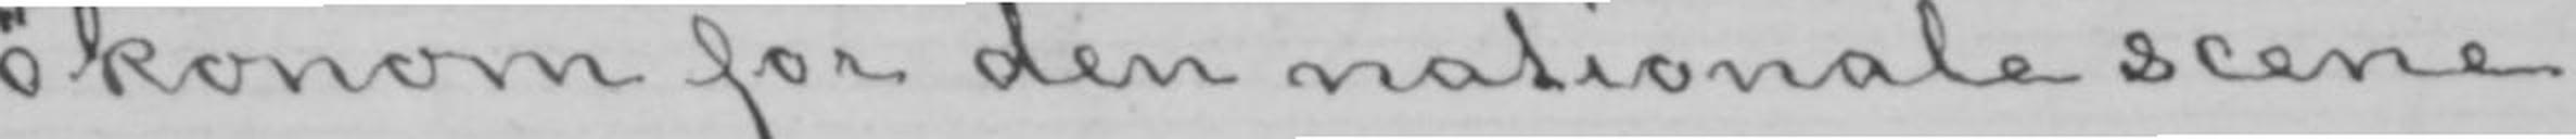økonom for den nationale scene
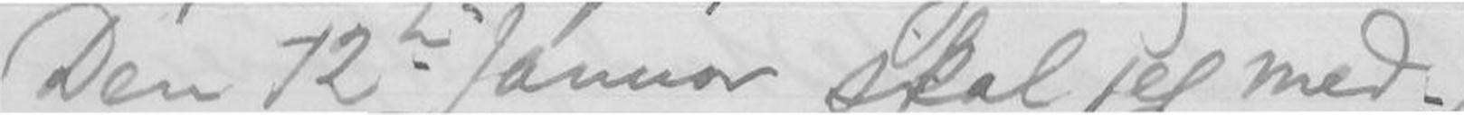Den 12te Januar skal jeg med-
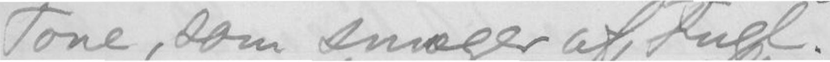Tone, som smager af Fugl.
